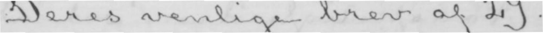Deres venlige brev af 29.
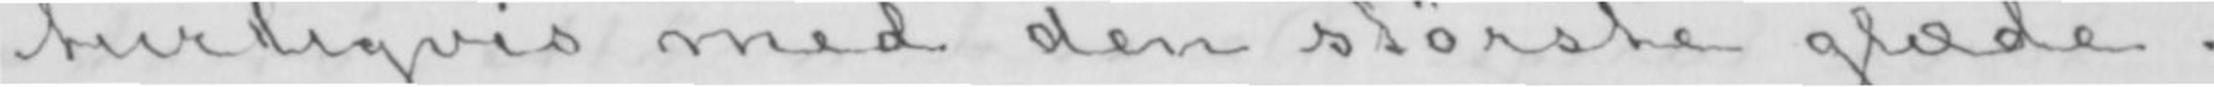turligvis med den største glæde.
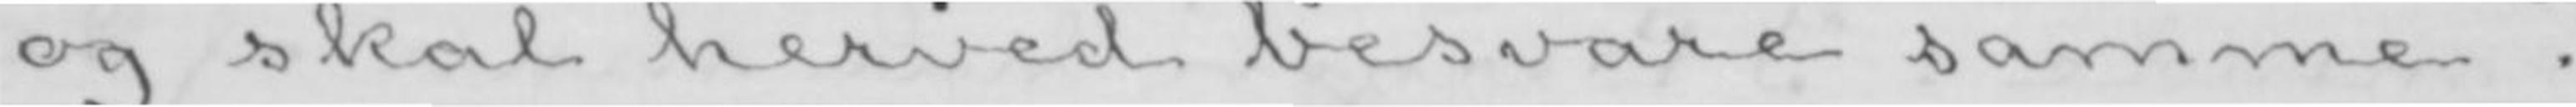og skal herved besvare samme.
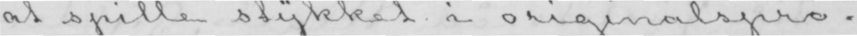at spille stykket i originalspro-
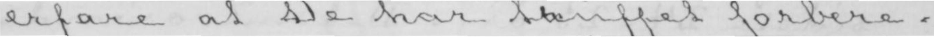erfare at De har turuffet forbere-
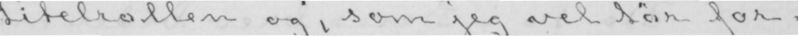titelrollen og, som jeg vel tør for-
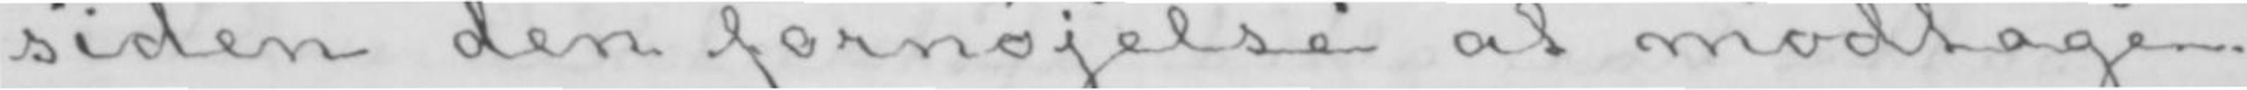siden den fornøjelse at modtage
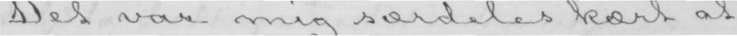Det var mig særdeles kært at
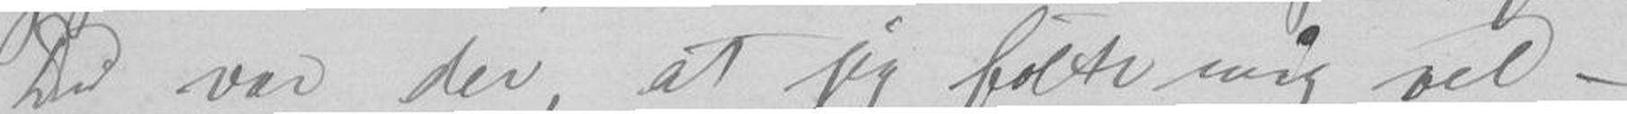Du var der, at jeg følte mig vel -
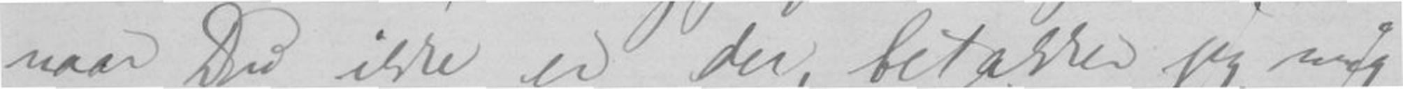naar Du ikke er der, betakker jeg mig
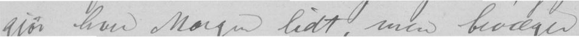gjør hver Morgen lidt, men bevæger
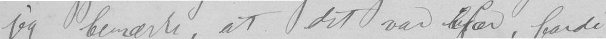jeg bemærke, at det var ufær, fordi
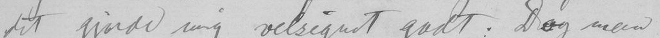det gjorde mig velsignet godt. Dog maa
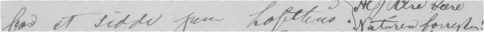for at sidde paa Lofthus. Naturen forresten!
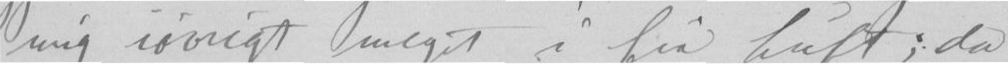mig iøvrigt meget i fri Luft; da
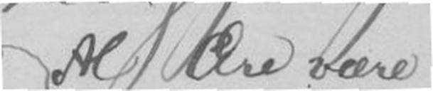Al Ære være
